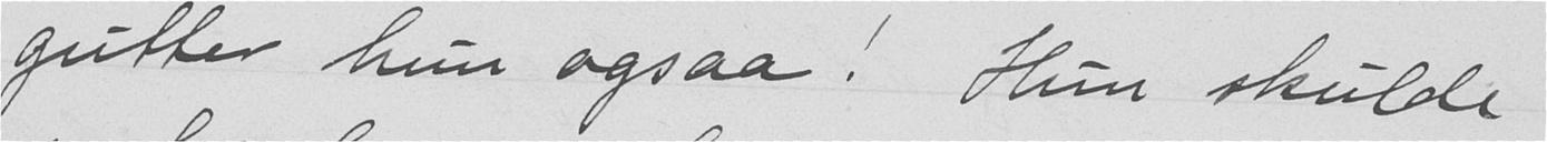gutter hun ogsaa! Hun skulde
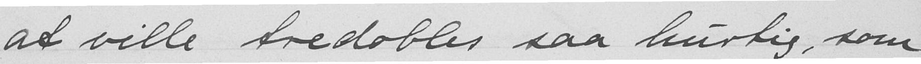at ville tredobles saa hurtig, som
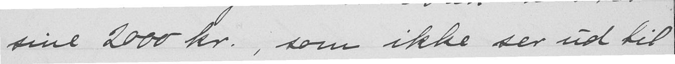sine 2000 kr., som ikke ser ud til
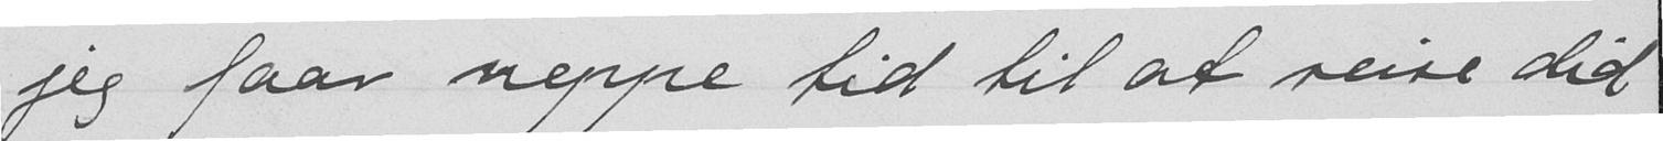jeg faar neppe tid til at reise did
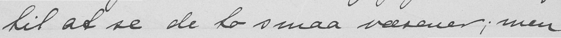til at se de to smaa væsener; men
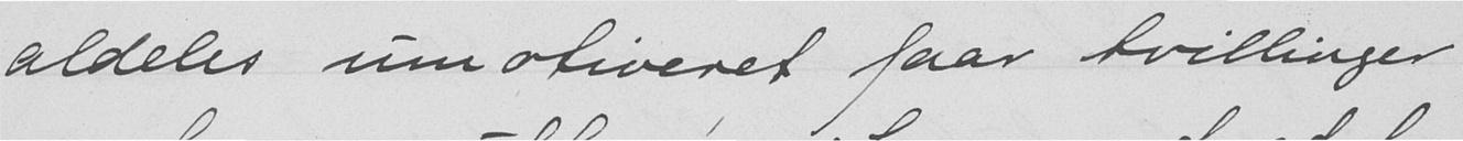aldeles umotiveret faar tvillinger
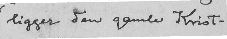ligger den gamle Krist-
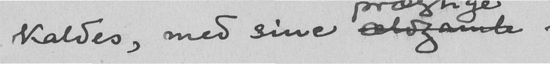kaldes, med sine ældgamle
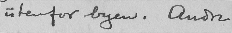utenfor byen. Andre
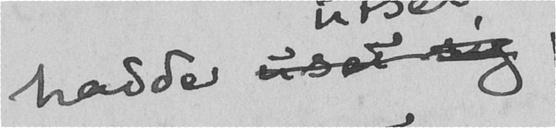hadde usat sig
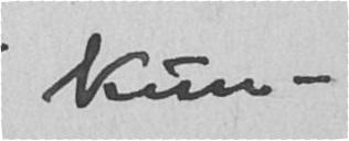kun-
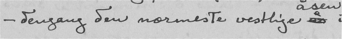- dengang den nærmeste vestlige å
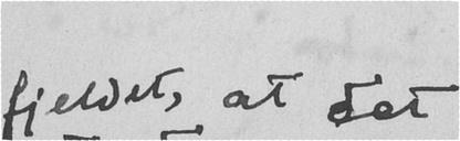fjeldet, at det
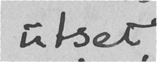utset
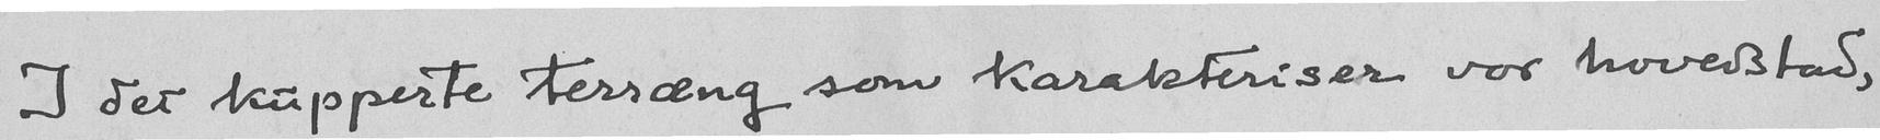I det kupperte terræng som karakteriser vor hovedstad,
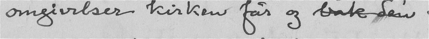omgivelser kirken får og bak den
